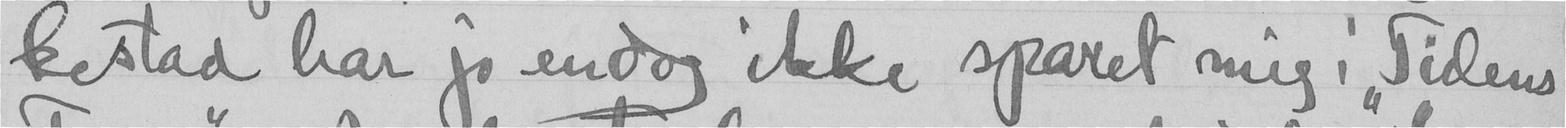kestad har jo endog ikke sparet mig i "Tidens
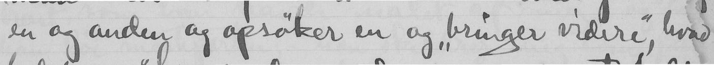en og anden og opsøker en og "bringer videre", hvad
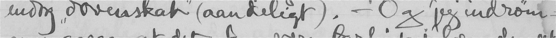endog "dovensskab" (aandeligt). - Og jeg indrøm-
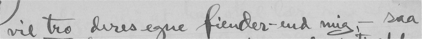vil tro deres egne fiender - end mig, - saa
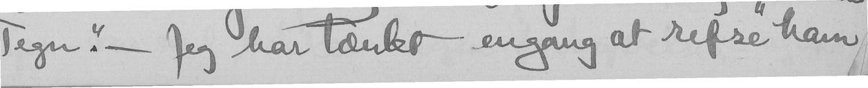Tegn". - Jeg har tænkt engang at refse ham
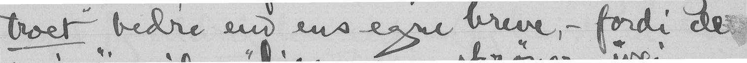troet bedre end ens egne breve, - fordi de
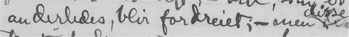anderledes, blir fordreiet; - men
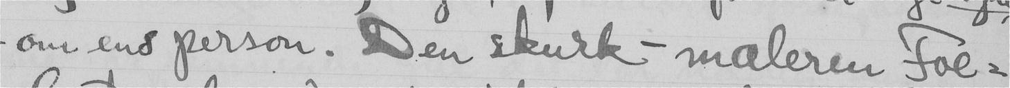om ens person. Den skurk - maleren Fol-
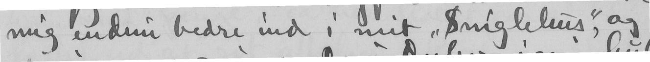mig endnu bedre ind i mit "sniglehus" og
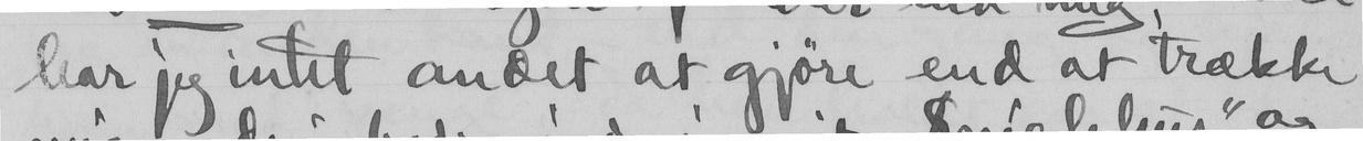har jeg intet andet at gjøre end at trække
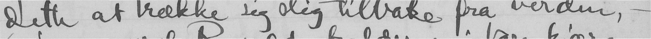dette at trække sig slig tilbake fra verden, -
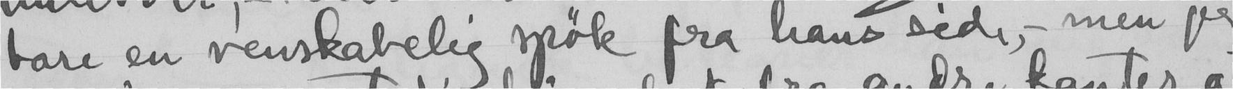bare en venskabelig spøk fra hans side, - men jeg
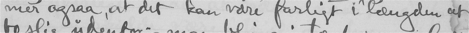mer ogsaa, at det kan være farligt i længden at
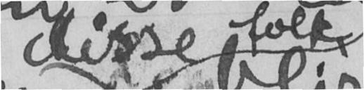Disse folk
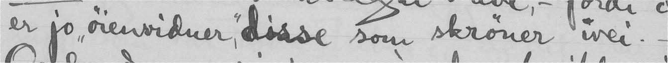er jo "øienvidner" disse som skrøner ivei. -
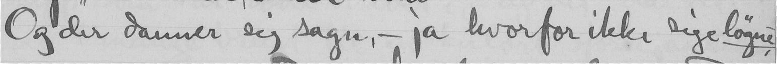Og der danner sig sagn, - ja hvorfor ikke sige løgne
